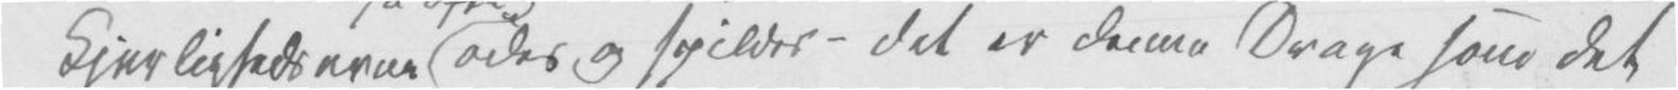Kjerlighedsevne ødes og spildes – det er denne Drage som det
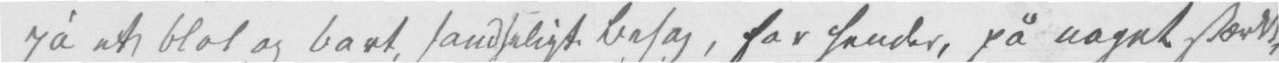på et blot og bart sandseligt Behag, for hendes, på noget stærkt,
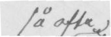så ofte
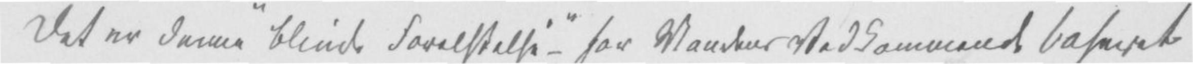Det er denne «blinde Forelskelse» – for Mandens Vedkommende baseret
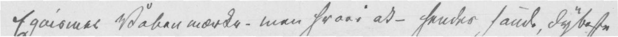Egoismes Våbenmærke – men hvori ak – hendes sande, dybeste
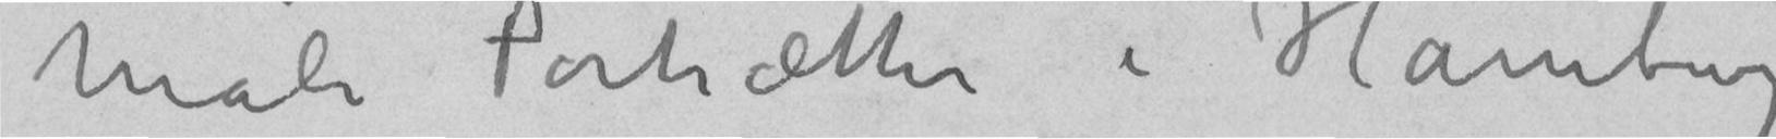male Portrætter i Hamburg
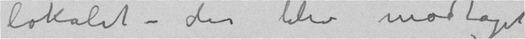lokalet – der blev modtaget
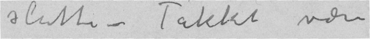slutte – Takket være
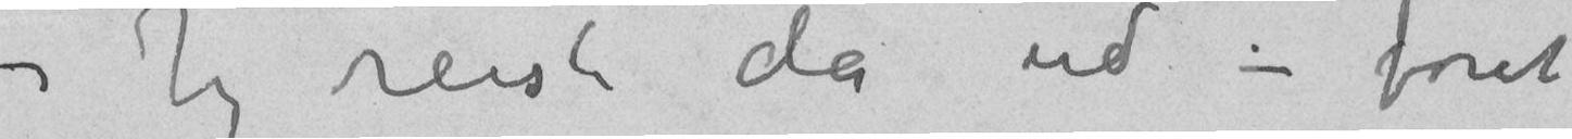– Jeg reiste da ud – forat
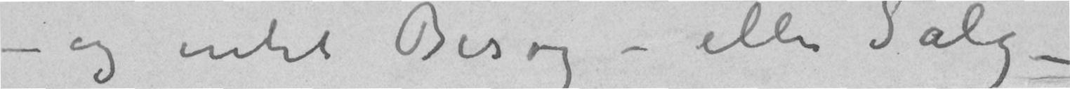– og intet Besøg – eller Salg –
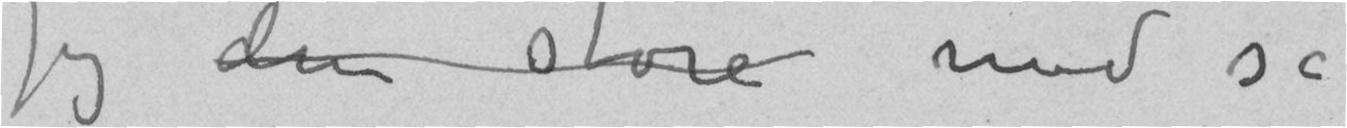jeg den store med så
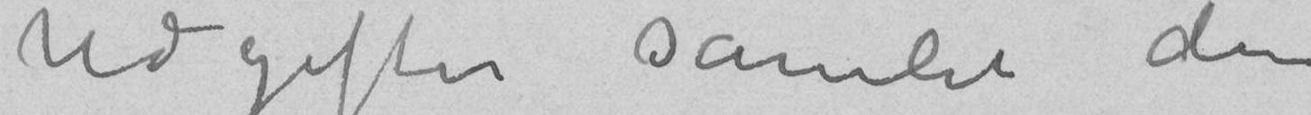Udgifter samlet den
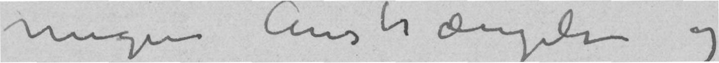megen Anstrængelse og
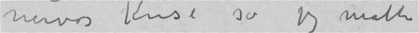nervøs Krise så jeg måtte
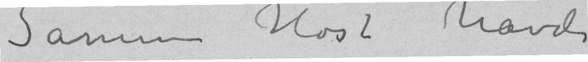Samme Høst havde
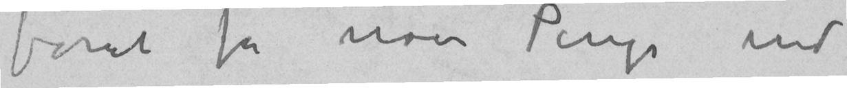forat få noen Penge ind
